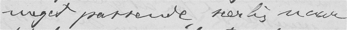meget passende, særlig naar
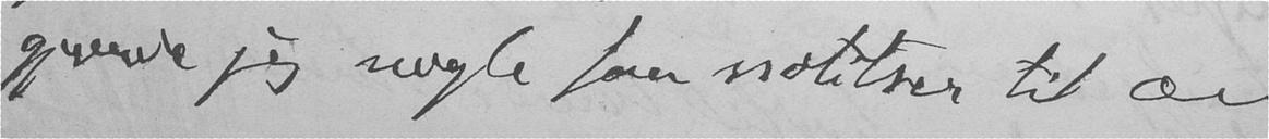gjorde jeg nogle faa notitser til æn-
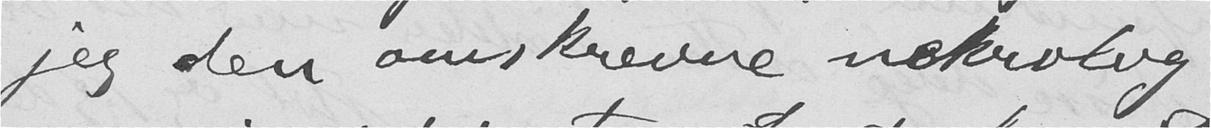jeg den omskrevne nekrolog,
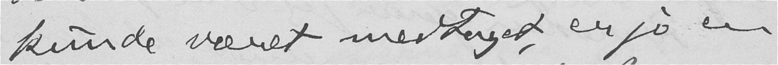kunde været medtaget, er jo en
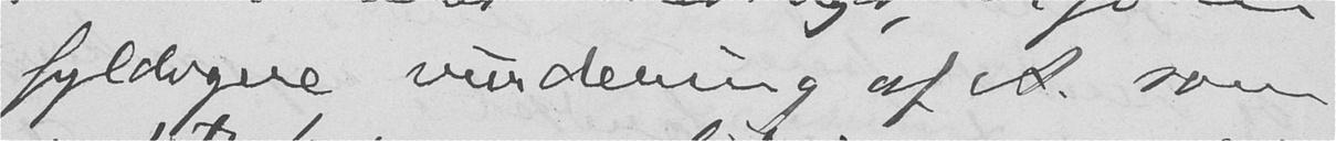fyldigere vurdering af A. som
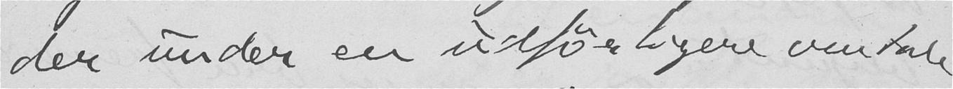der under en udførligere omtale
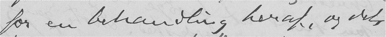for en behandling heraf, og dels
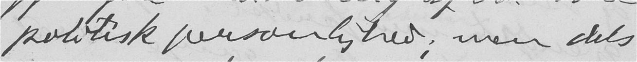politisk personlighed; men dels
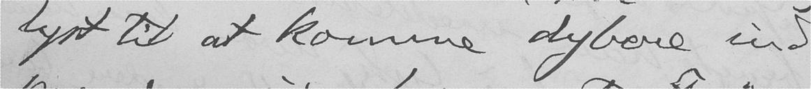lyst til at komme dybere ind
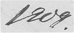1909.
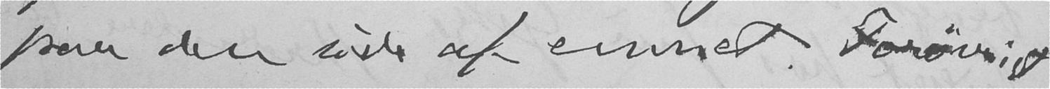paa den side af emnet. Forøvrigt
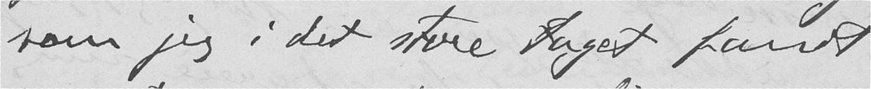som jeg i det store taget fandt
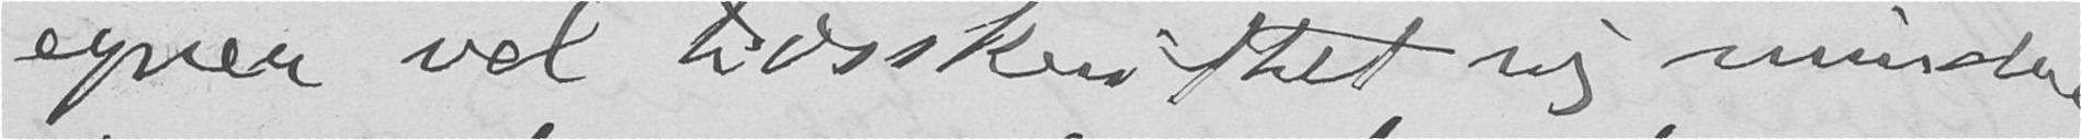egner vel tidsskriftet sig mindre
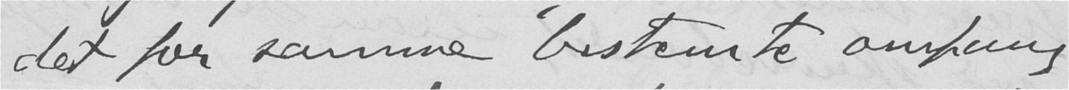det for samme bestemte omfang
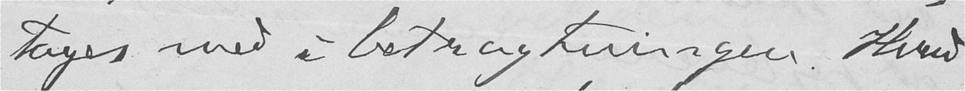tages med i betragtningen. Hvad
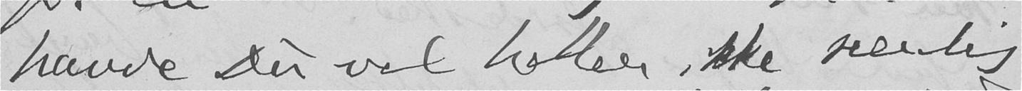havde Du vel heller ikke særlig
